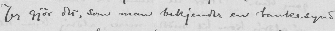Jeg gjør det, som man bekjender en tankesynd
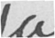ja
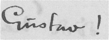Gustav!
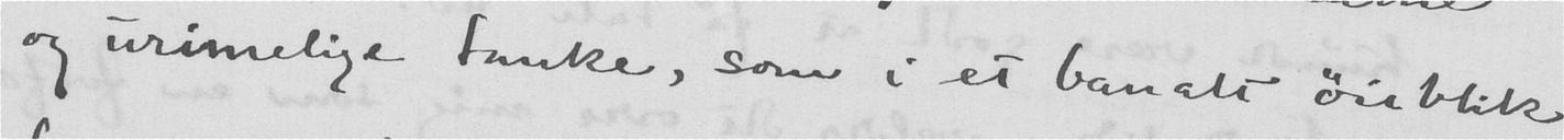og urimelige tanke, som i et banalt øieblik
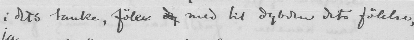i dets tanke, føler dy med til dybden dets følelse,
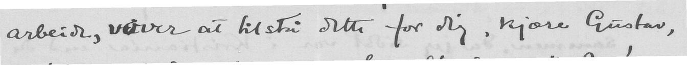arbeide, vover at tilstå dette for dig, kjære Gustav,
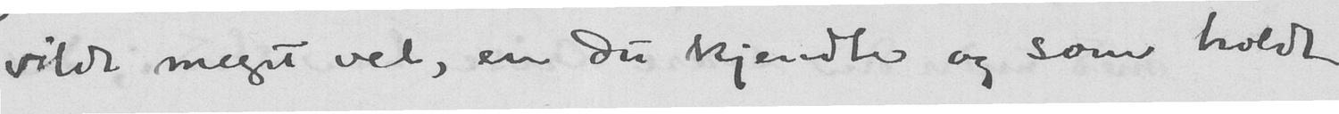vilde meget vel, en du kjendte og som holdt
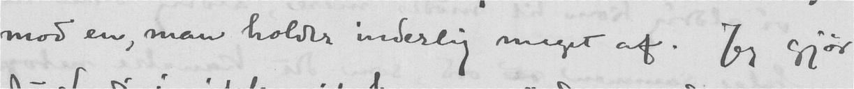mod en man holder inderlig meget af. Jeg gjør
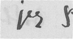jeg
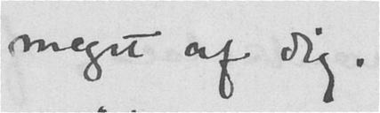meget af dig.
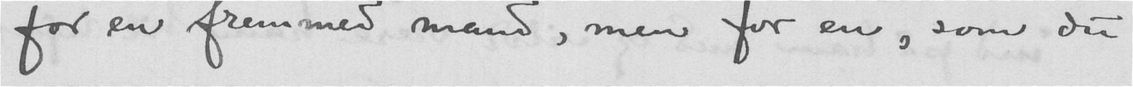for en fremmed mand, men for en, som du
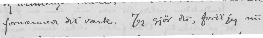fornærmede det værk. Jeg gjør det, fordi jeg nu
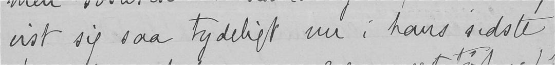vist sig saa tydeligt nu i hans sidste
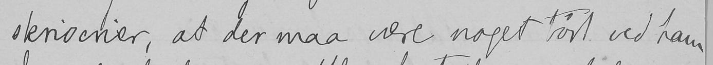skriverier, at der maa være noget tørt ved ham
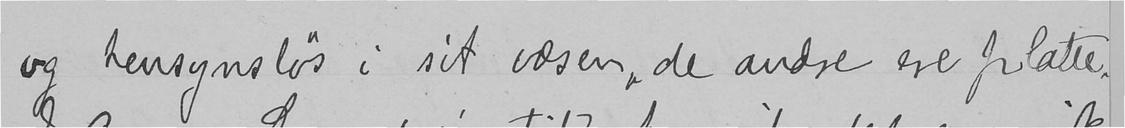og hensynsløs i sit væsen, de andre ere platte.
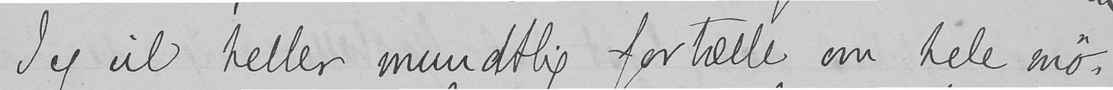Jeg vil heller mundtlig fortælle om hele mø-
